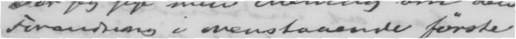Forandring i ovenstaaende første
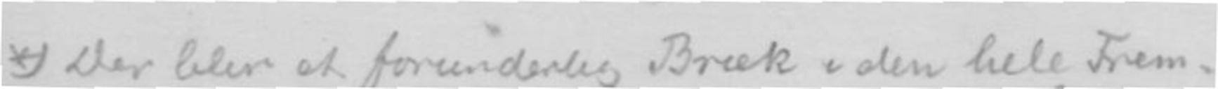x) Der blev etforunderlig Bræk i den hele Frem-
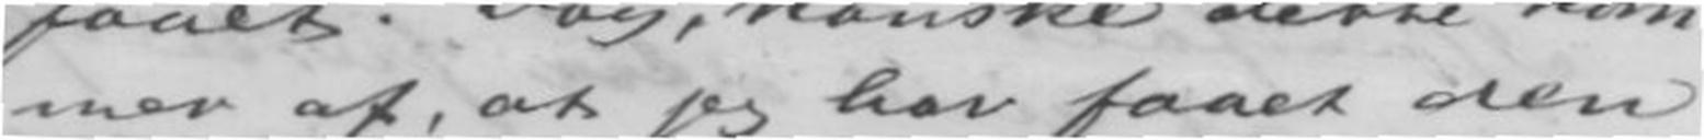me af, at jeg har faaet den
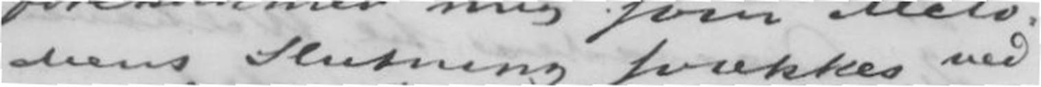diens Slutning svækkes ved
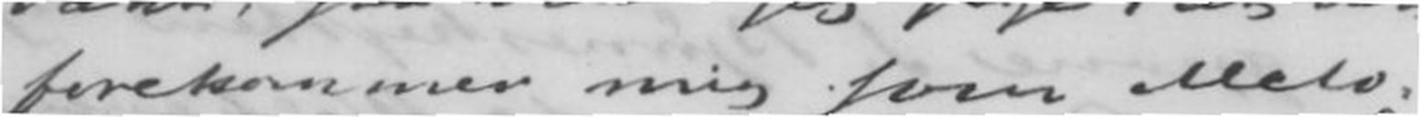forekommer mig som Melo
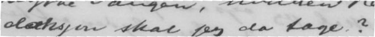daksjon skal jeg da tage?
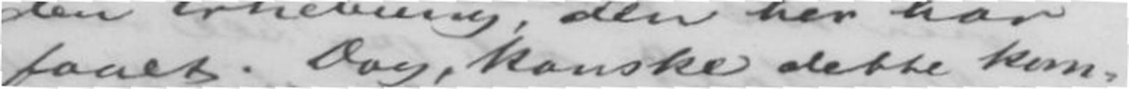faaet. Dog, kanske dette kom
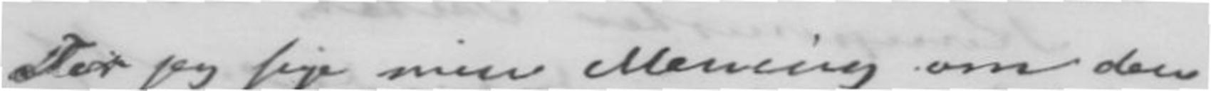Der jeg sige min Mening om den
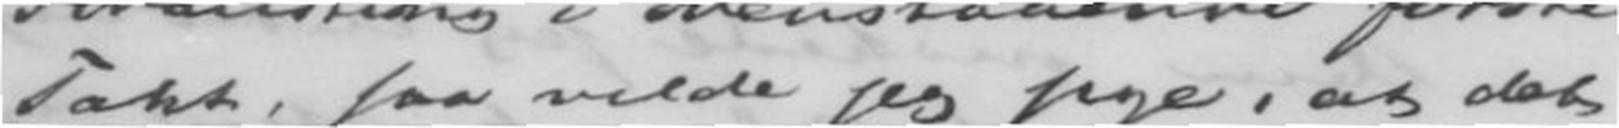Takt, saa vilde jeg sige, at det
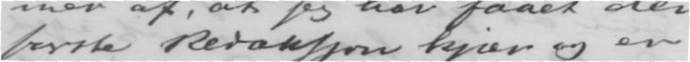første Redaksjon kjær og er
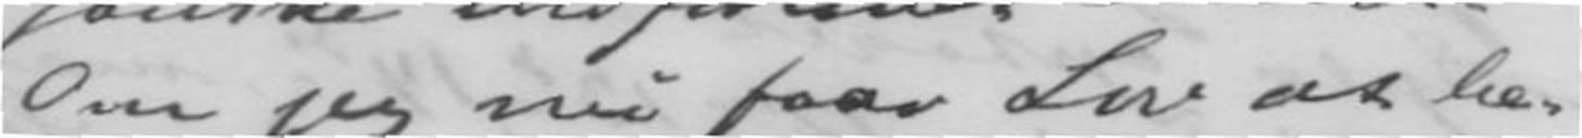Om jeg nu faar Lov at be-
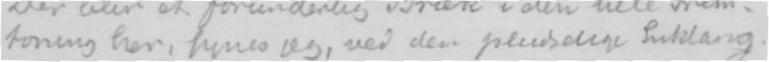toning her, synes jeg, ved den pludselige Inklang
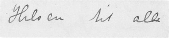Hilsen til alle
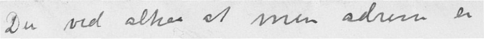Du ved altsaa at min adresse er
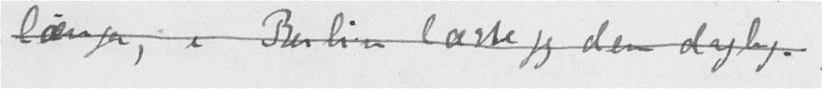længer, i Berlin læste jeg dem daglig.
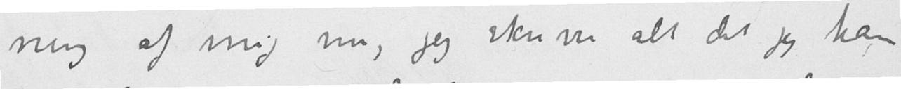ning af mig nu, jeg skriver alt det jeg kan
